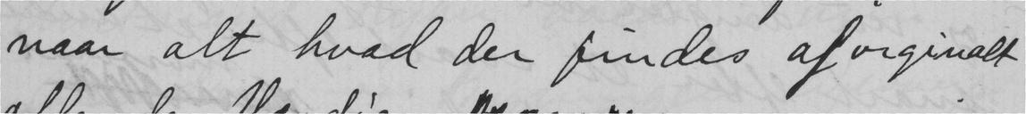naar alt hvad der findes af orginalt
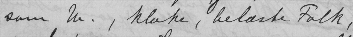som W., kloke, belæste Folk,
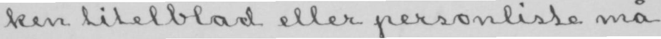ken titelblad eller personliste må
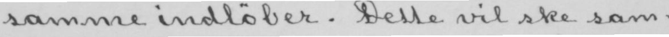samme indløber. Dette vil ske sam-
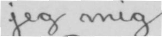jeg mig
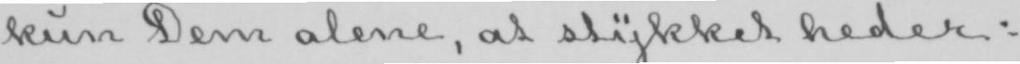kun Dem alene, at stykket heder:
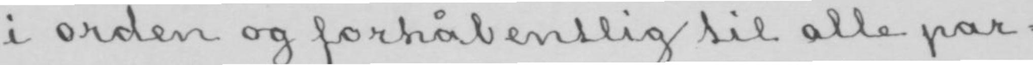i orden og forhåbentlig til alle par-
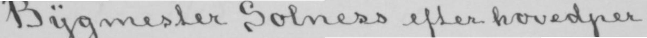Bygmester Solness efter hovedper-
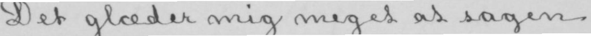Det glæder mig meget at sagen
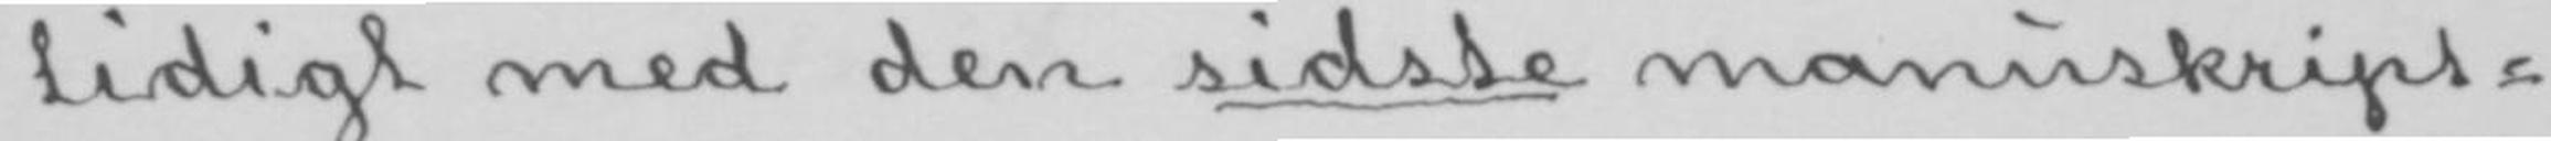tidigt med den sidste manuskript-
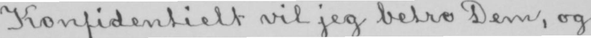Konfidentielt vil jeg betro Dem, og
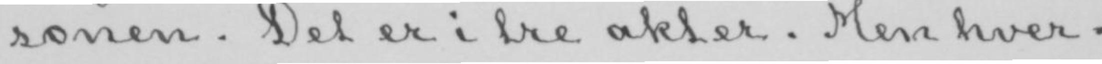sonen. Det er i tre akter. Men hver-
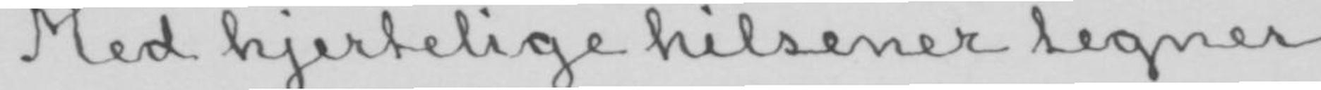Med hjertelige hilsener tegner
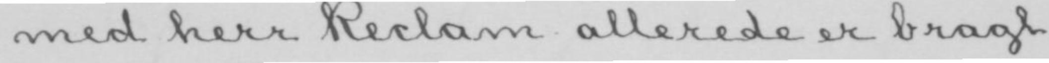med herr Reclam allerede er bragt
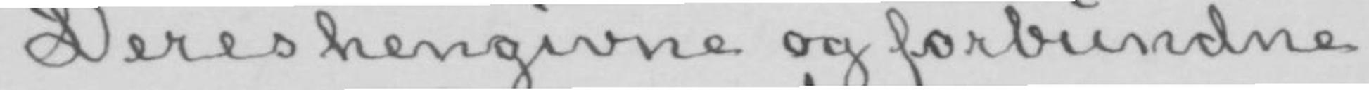Deres hengivne og forbundne
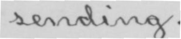sending.
